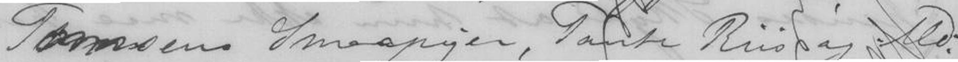Tomsens Smaapiger, Tante Riis og Md:
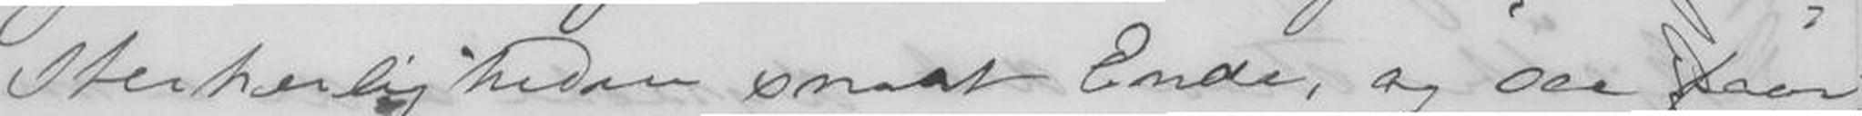sterherligheden snart Ende, og saa faar
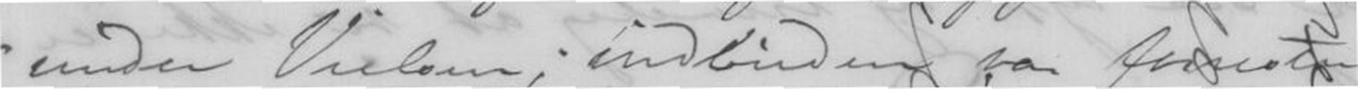under Vielsen; indbuden var forresten
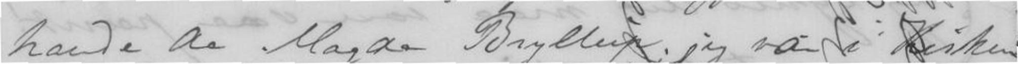havde da Magda Bryllup; jeg var i Kirken
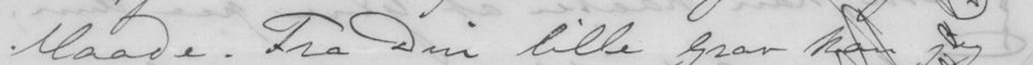Maade. Fra Din lille Grav kan jeg
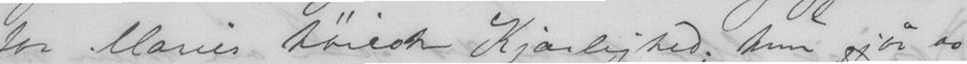for Maries høieste Kjærlighed; hun gjør os
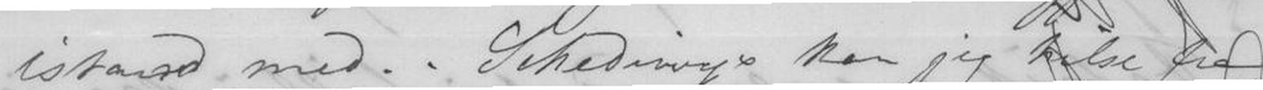istand med. Schediwys kan jeg hilse fra;
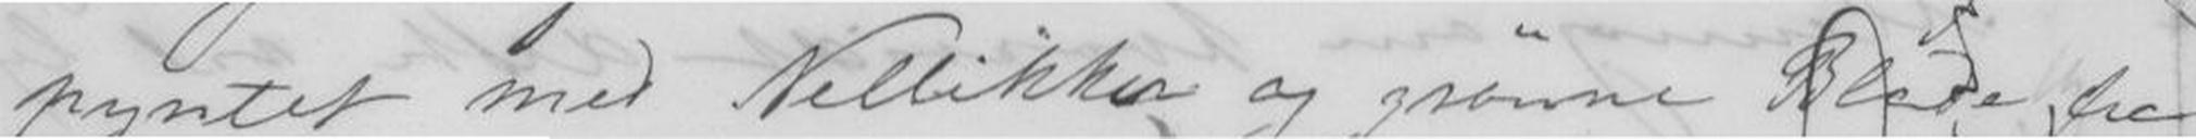pyntet med Nellikker og grønne Blade fra
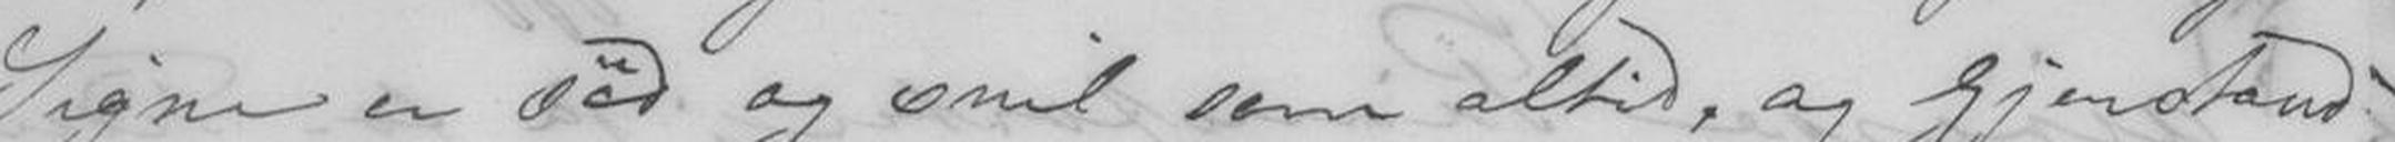signe er sød og snil som altid, og Gjenstand
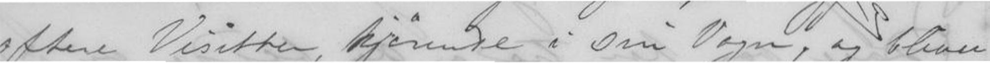oftere Visitter, kjørende i sin Vogn, og bliver
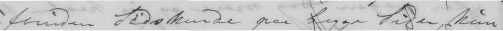foruden Sødskende paa begge Sider, kun
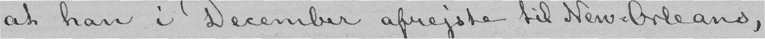at han i December afrejste til New-Orleans,
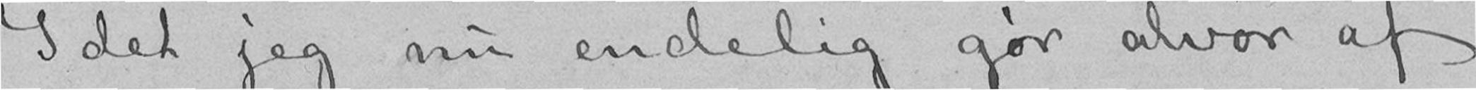Idet jeg nu endelig gør alvor af
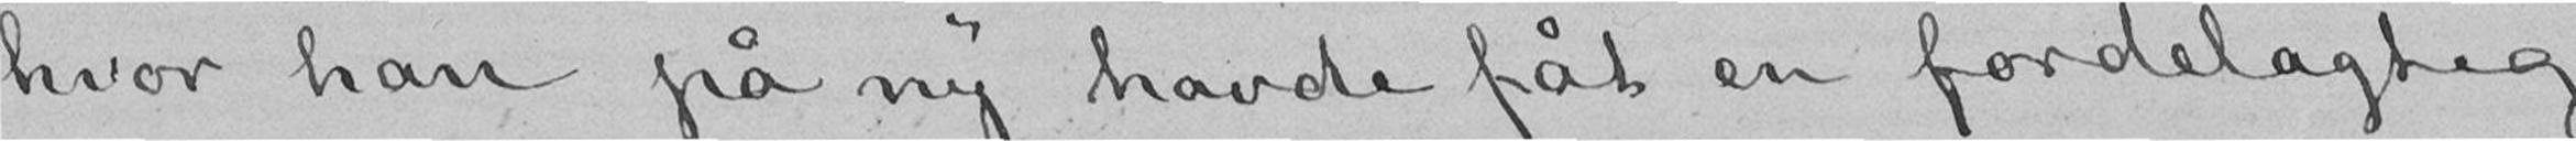hvor han på ny havde fåt en fordelagtig
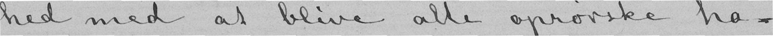hed med at blive alle oprørske ho-
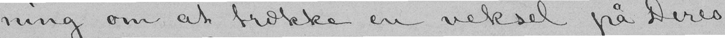ning om at trække en veksel på Deres
